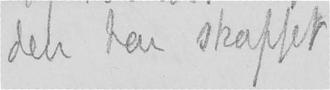den har skaffet
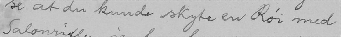se at du kunde skyte en Røi med
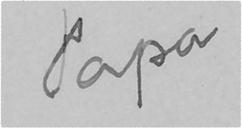Papa
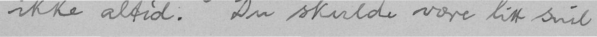ikke altid. Du skulde være litt snil
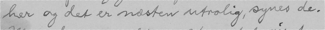her og det er næsten utrolig, synes de.
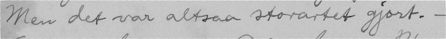Men det var altsaa storartet gjort. -
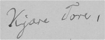Kjære Tore,
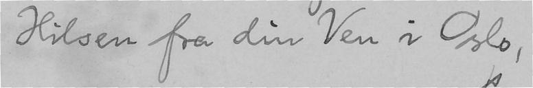Hilsen fra din Ven i Oslo,
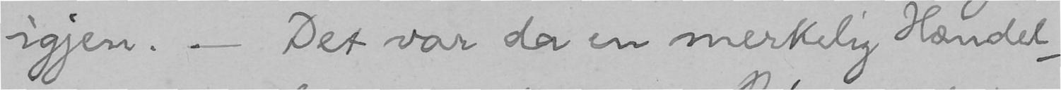igjen. - Det var da en merkelig Hændel-
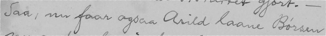Saa, nu faar ogsaa Arild laane Børsen
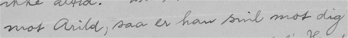mot Arild, saa er han snil mot dig
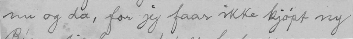nu og da, for jeg faar ikke kjøpt ny
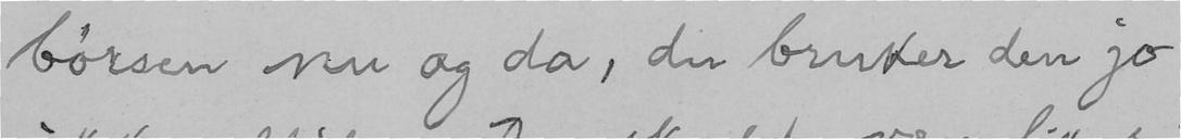børsen nu og da, du bruker den jo
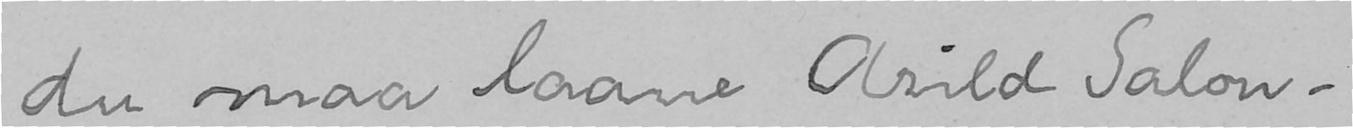du maa laane Arild Salon-
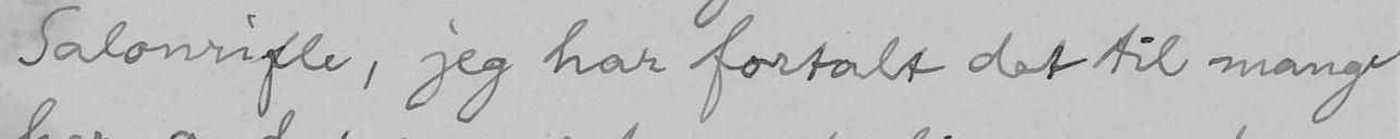Salonrifle, jeg har fortalt det til mange
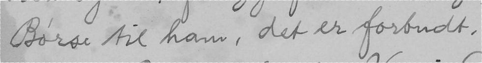Børse til ham, det er forbudt.
